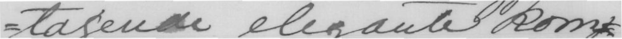tagende elegante kom-
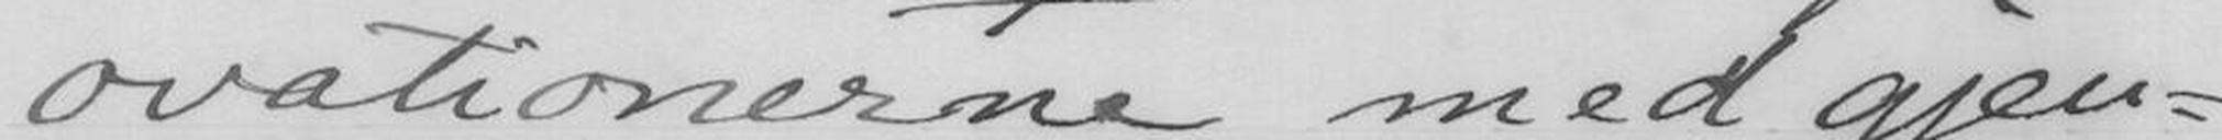ovationerne med gjen-
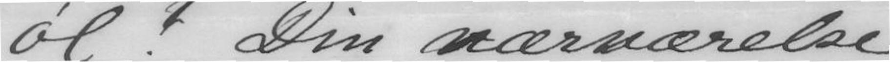øl? Din nærværelse
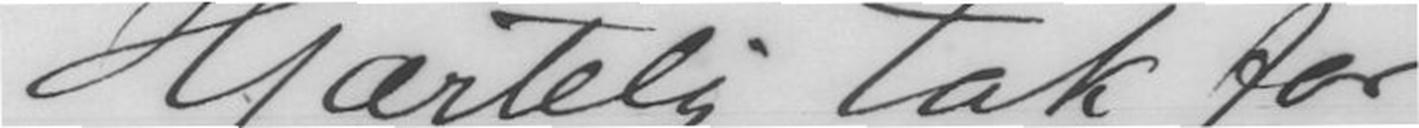Hjærtelig tak for
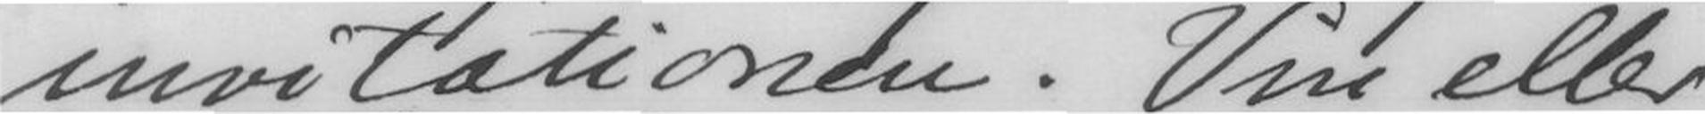invitationen. Vin eller
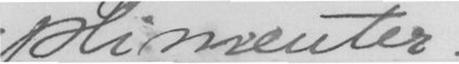plimenter.
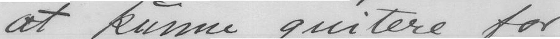at kunne quitere for
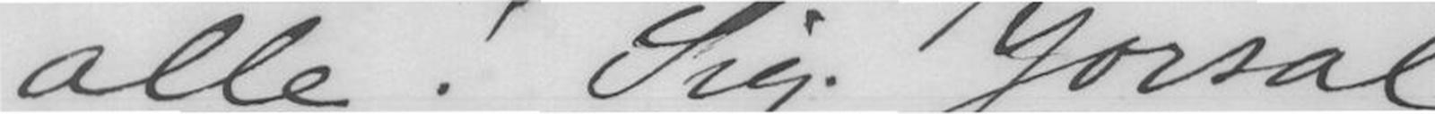alle! Sig. Jorsal
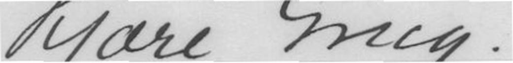Kjære Grieg!
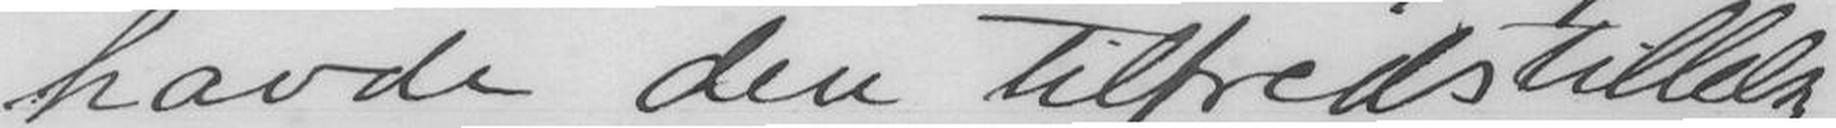havde den tilfredstillelse
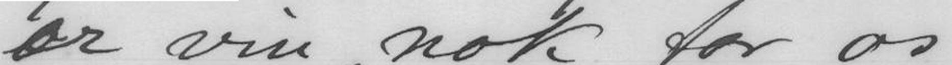er vin nok for os
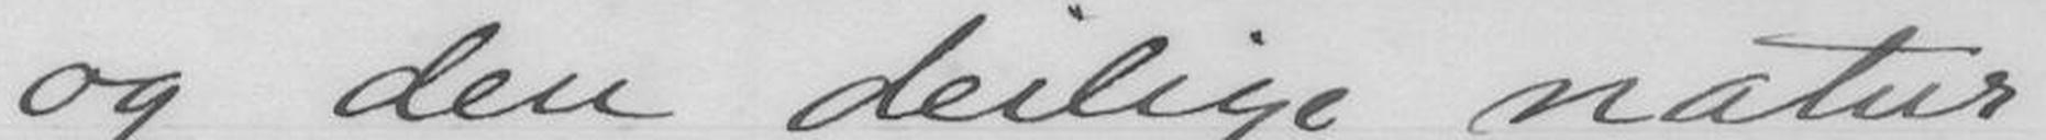og den deilige natur
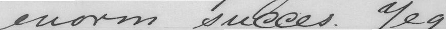enorm succes. Jeg
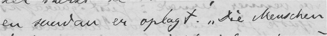en saadan er oplagt. "Die Menschen
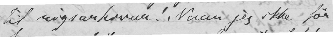til rigsarkivar! Naar jeg ikke før
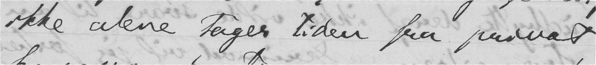ikke alene tager tiden fra privat
